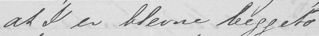at I er blevne beggeto
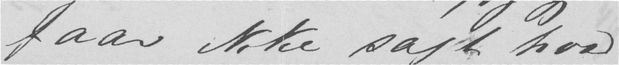faar ikke sagt hvad
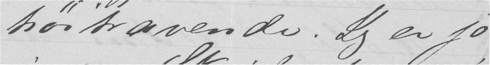høitravende. Jeg er jo
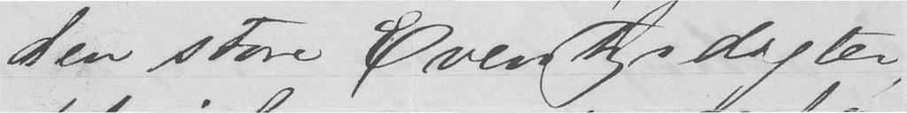den store Eventyrdigter,
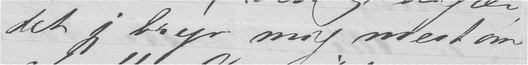det jeg bryr mig mest om
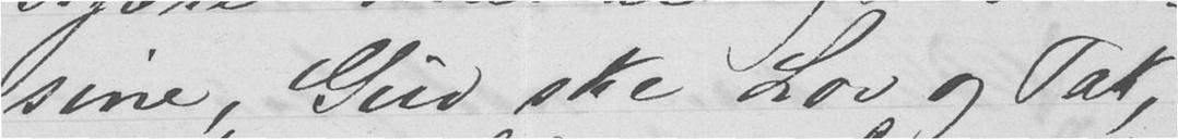sine, Gud ske Lov og Tak,
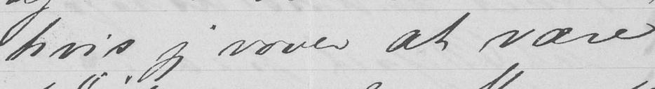hvis jeg vover at være
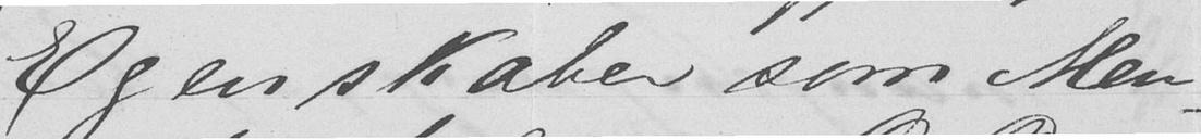Egenskaber som Men-
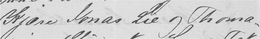Kjære Jonas Lie og Thoma-
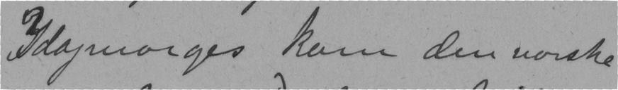Idagmorges kom den norske
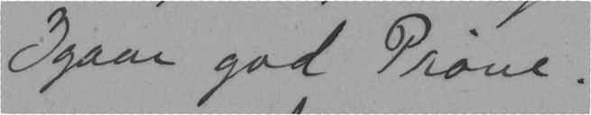Igaar god Prøve.
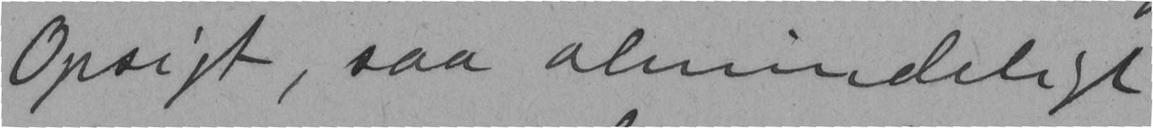Opsigt, saa almindeligt
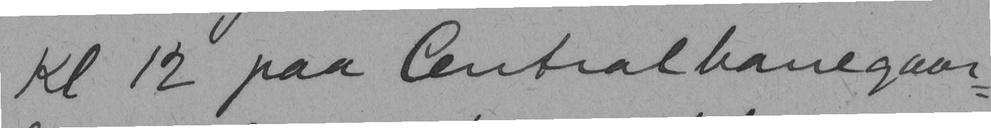Kl 12 paa Centralbanegaar-
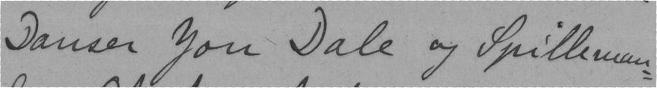Danser Jon Dale og Spilleman-
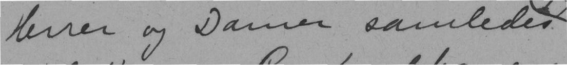Herrer og Damer samledes
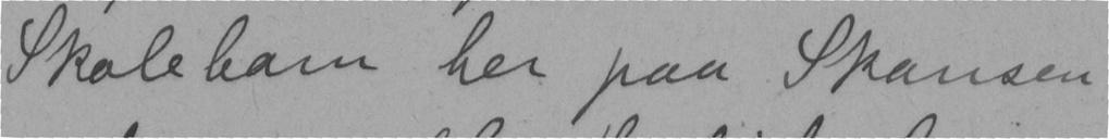Skolebarn her paa Skansen
